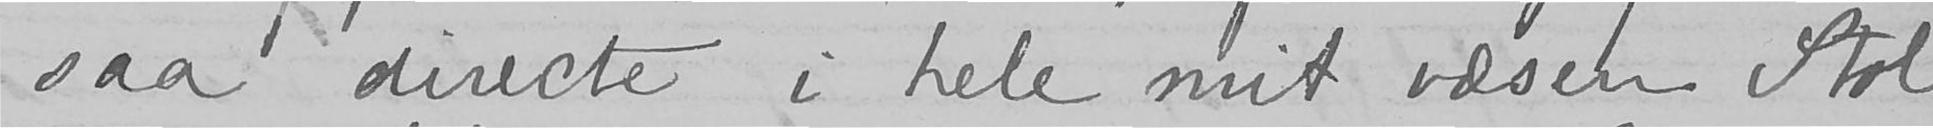saa directe i hele mit væsen. Stol
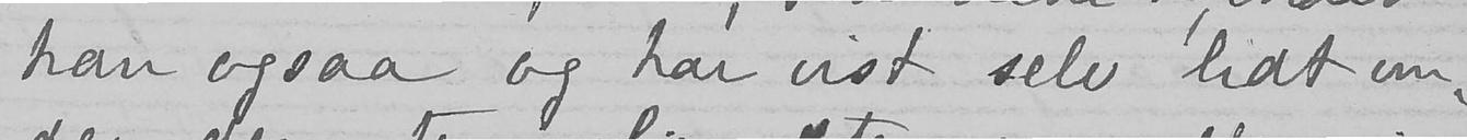han ogsaa og har vist selv lidt un-
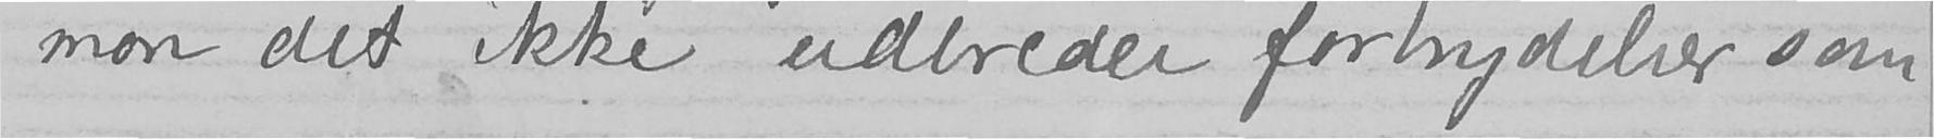mon det ikke udbreder forbrydelser som
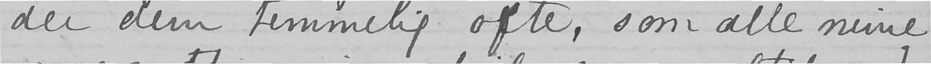der dem temmelig ofte, som alle mine
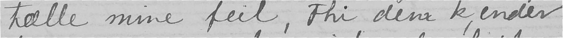tælle mine feil, thi dem kjender
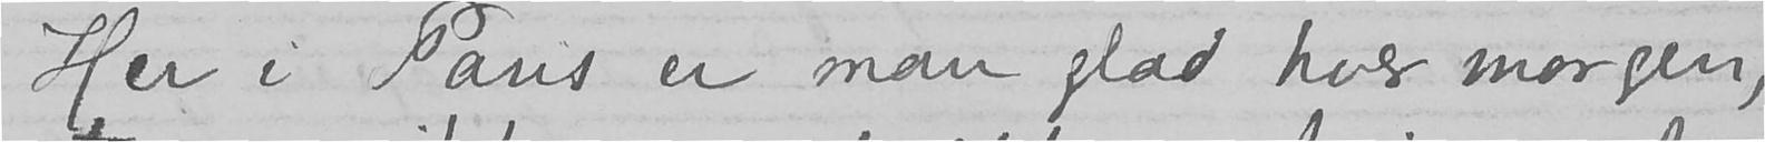Her i Paris er man glad hver morgen,
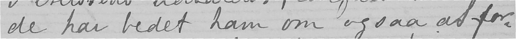de har bedet ham om ogsaa at for-
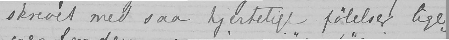skrevet med saa hjertelige følelser lige-
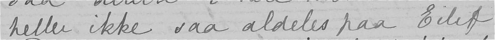heller ikke saa aldeles paa, Eilif
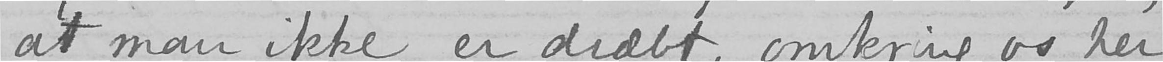at man ikke er dræbt, omkring os her-
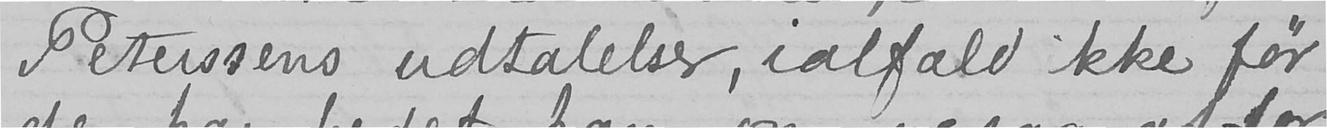Peterssens udtalelser, ialfald ikke før
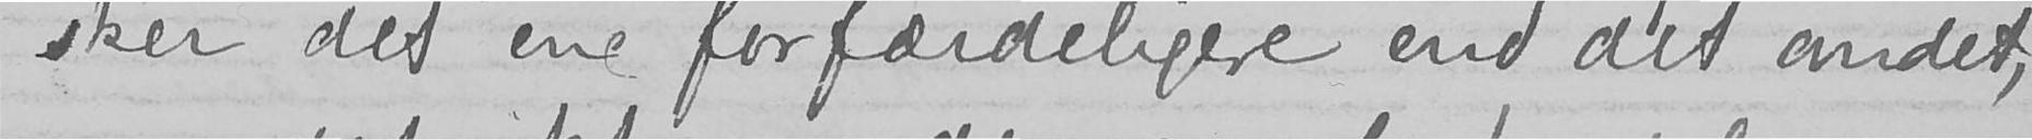sker det ene forfærdeligere end det andet,
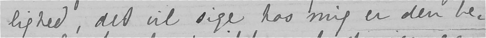lighed, det vil sige hos mig, er den be-
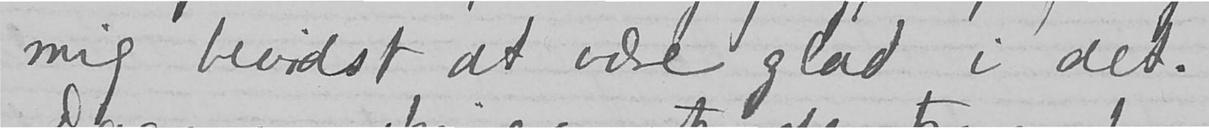mig bevidst at være glad i det.
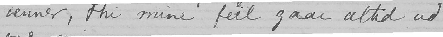venner, thi mine feil gaar altid ud
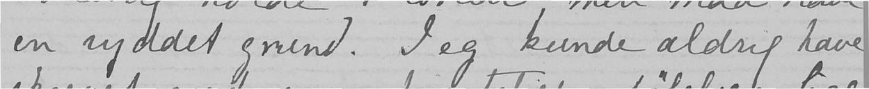en ryddet grund. Jeg kunde aldrig have
